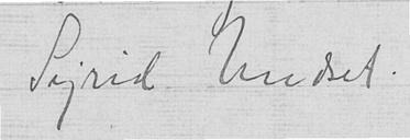Sigrid Undset.
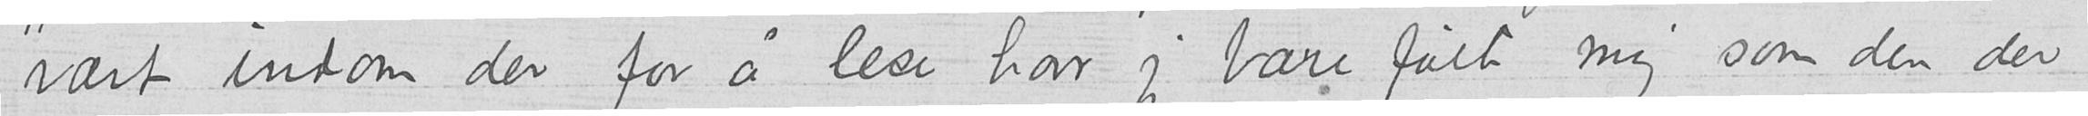vært indom der for å lese har jeg bare følt mig som den der
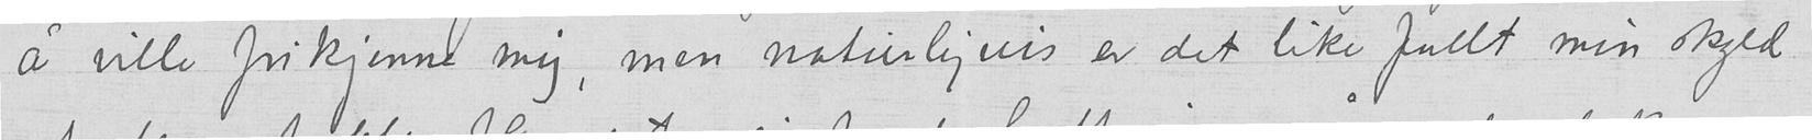å ville frikjenne mig, men naturligvis er det like fullt min skyld
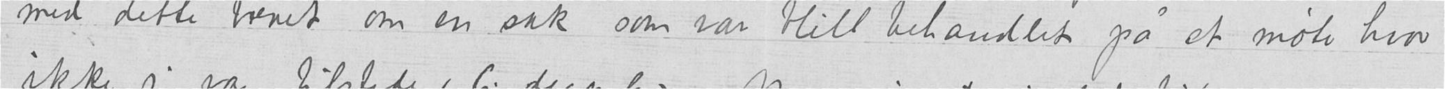med dette brevet om en sak som var blitt behandlet pa et møte hvor
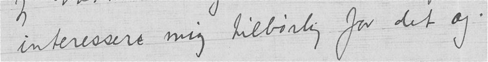interessere mig tilbørlig for det og.
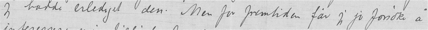jeg hadde erlediget den. Men for fremtiden får jeg jo forsøke å
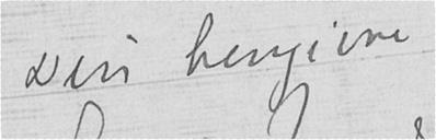Din hengivne
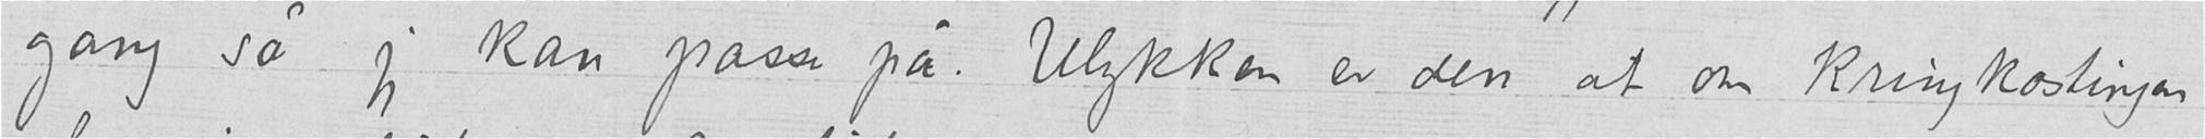gang så jeg kan passe på. Ulykken er den at om Kringkastingen
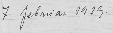7. februar 1929.
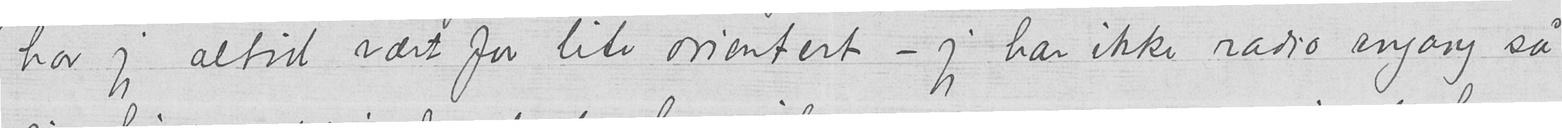har jeg altid vært for lite orientert – jeg har ikke radio engang så
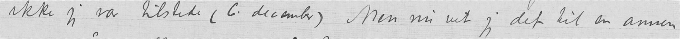ikke jeg var tilstede (6. december) Men nu vet jeg det til en annen
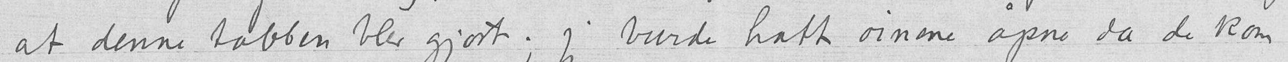at denne tabben blev gjort; jeg burde hatt øinene åpne da de kom
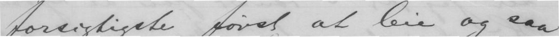forsigtigste først at leie og saa
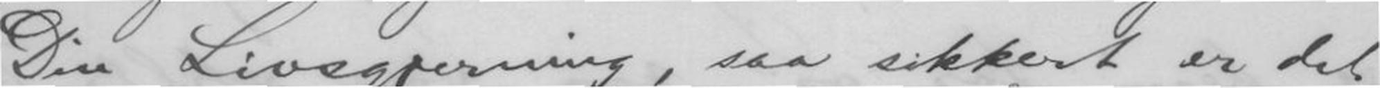Din Livsgjerning, saa sikkert er det
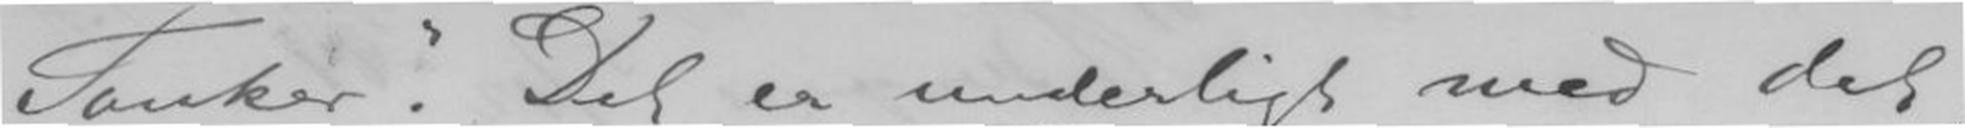Tanker". Det er underligt med det,
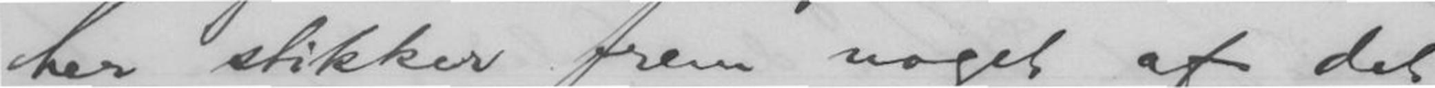her stikker frem noget af det
